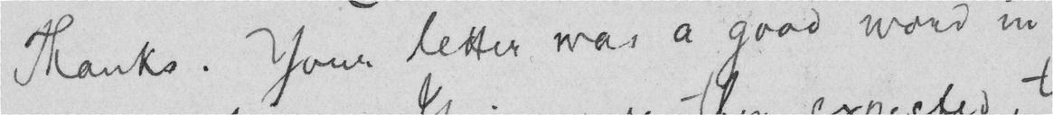Thanks. Your letter was a good word in
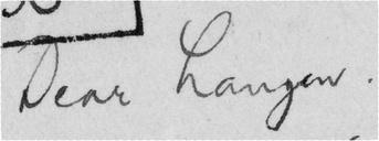Dear Langen.
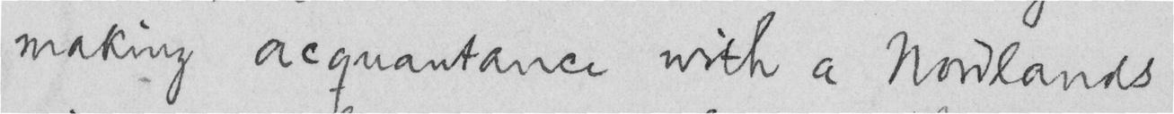making acquantance with a Nordlands
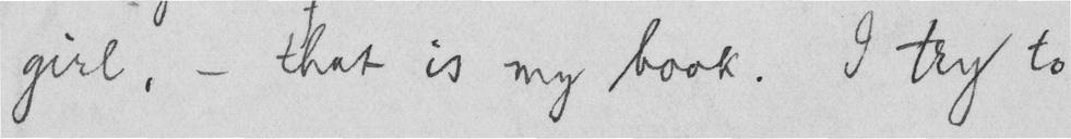girl, - that is my book. I try to
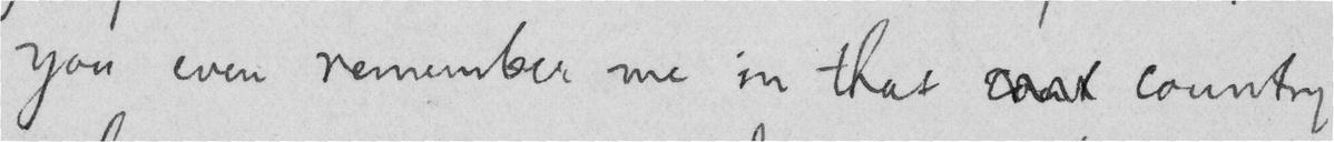you even remember me in that cont country
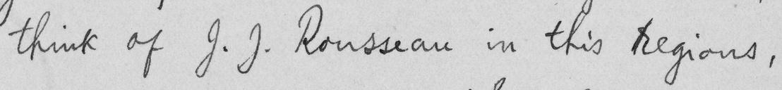think of J. J. Rousseau in this regions,
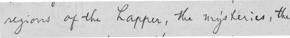regions of the Lapper, the mysteries, the
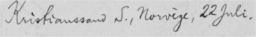Kristianssand S., Norvège, 22 Juli.
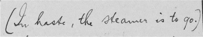(In haste, the steamer is to go.)
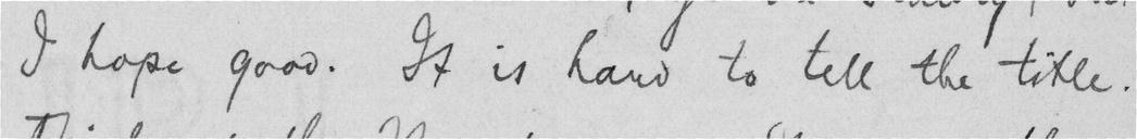I hope good. It is hard to tell the title.
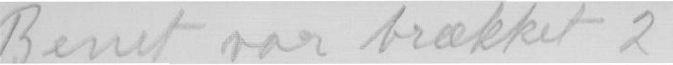Benet var brækket 2
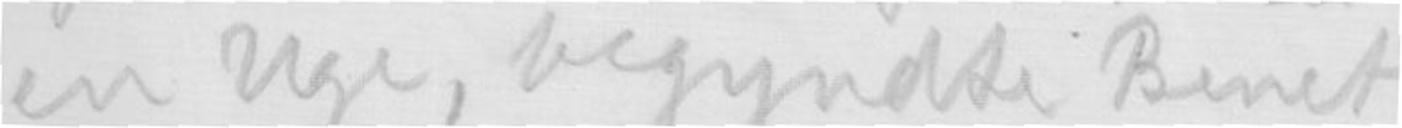en Uge, begyndte Benet
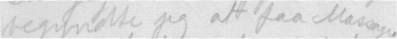begyndte jeg at faa Massage,
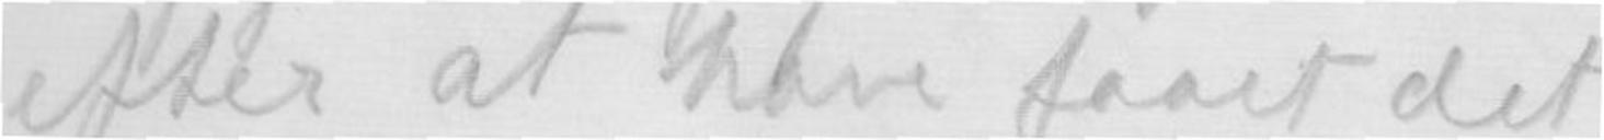efter at have faaet det
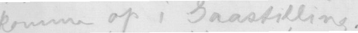komme op i Gaastilling.
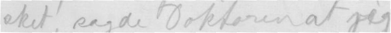sket, sagde Doktoren at jeg
